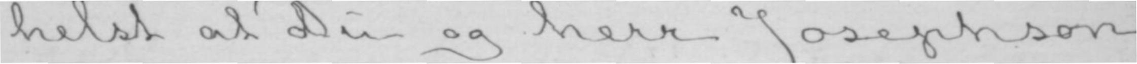helst at dDu og herr Josephson
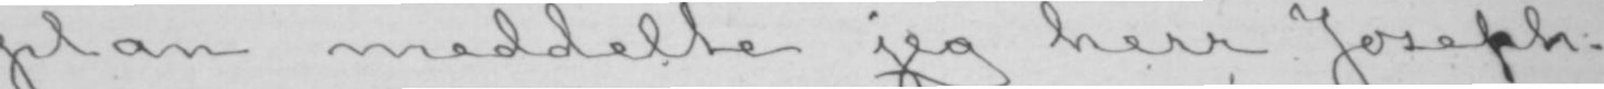plan meddelte jeg herr Joseph-
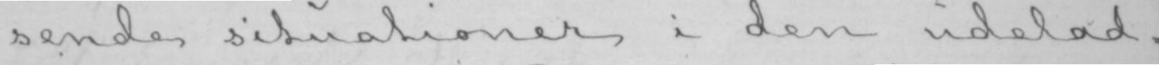sende situationer i den udelad-
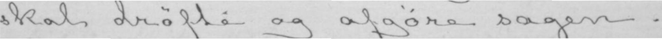skal drøfte og afgøre sagen.
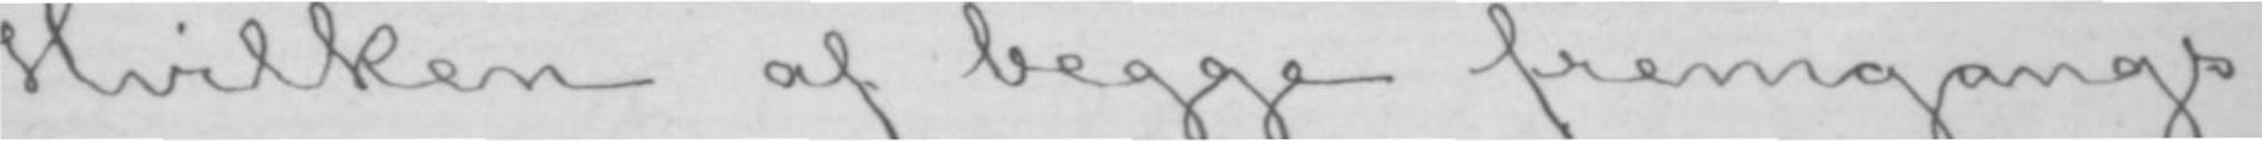Hvilken af begge fremgangs-
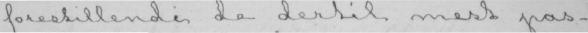forestillende de dertil mest pas-
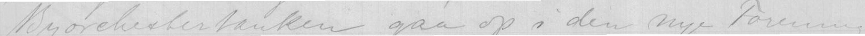Byorchestertanken, gaa op i den nye Forening
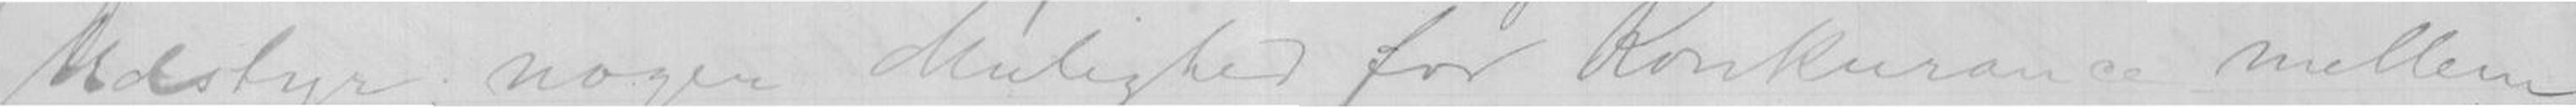Udstyr; nogen Mulighed for Konkurance mellem
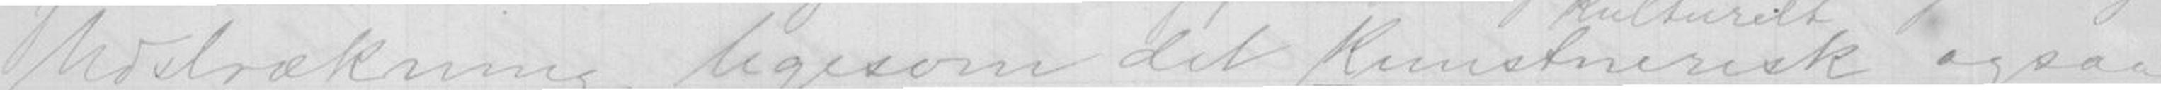Udstrækning, ligesom det kunstnerisk ogsaa
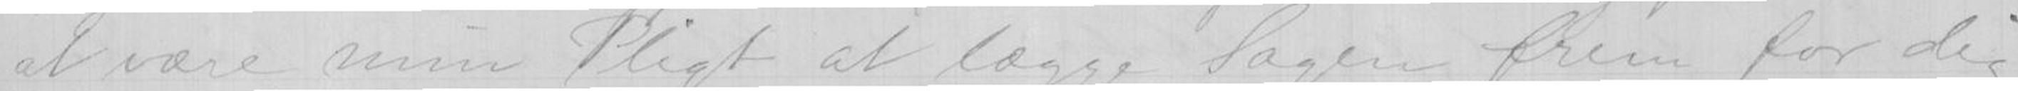at være min Pligt at lægge Sagen frem for dig
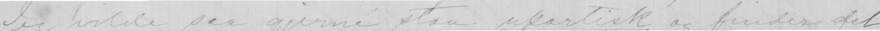Jeg vilde saa gjerne staa upartisk og finder det
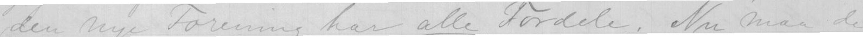den nye Forening har alle Fordele. Nu maa de
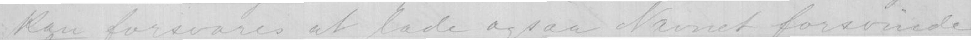kan forsvares at lade ogsaa Navnet forsvinde
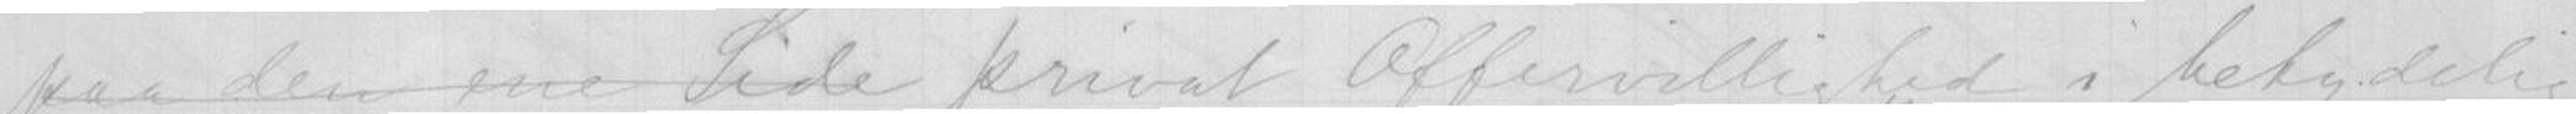paa den ene Side privat Offervillighed i betydelig
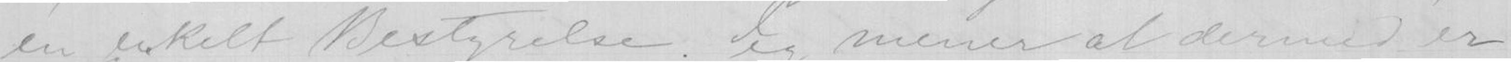en enkelt Bestyrelse. Jeg mener at dermed er
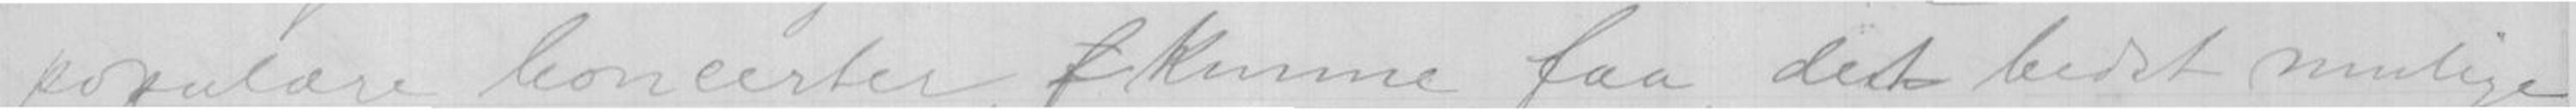populære Concerter f kunne faa det bedst mulige
